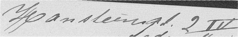Hansteensgt. 2 IV
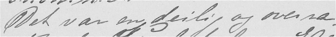Det var en deilig og overra-
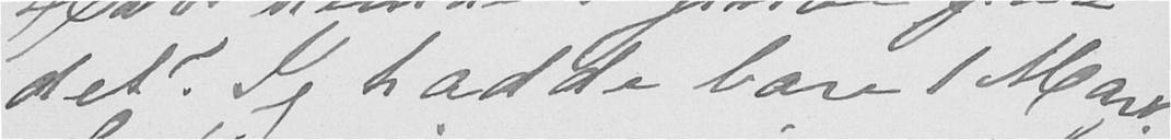det? Jeg hadde bare1 Mark
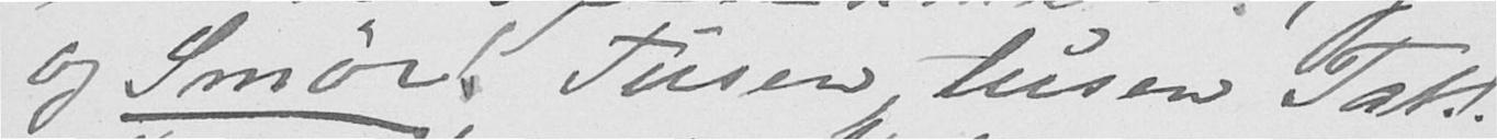og Smør. Tusen, tusen Takk
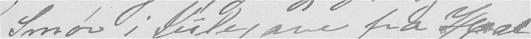Smør i Julegave fra Hal-
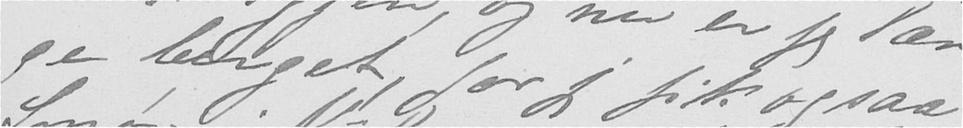ge berget, for jeg fik ogsaa
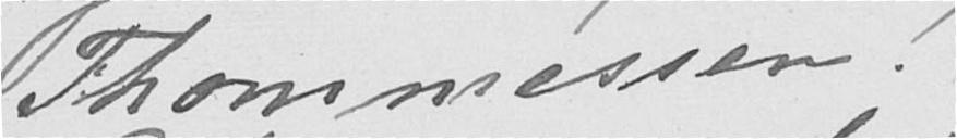Thommessen.
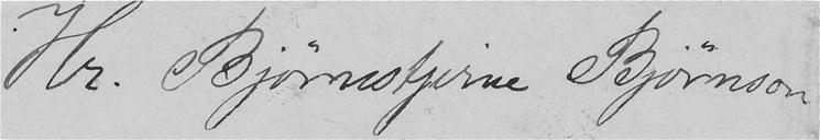Hr. Bjørnstjerne Bjørnson
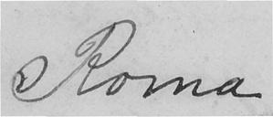Roma
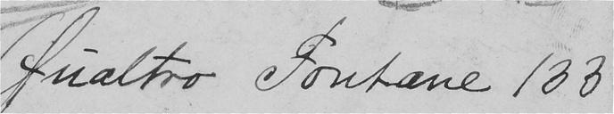Qualtro Fontane 133
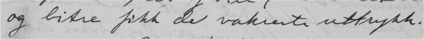og bitre fikk de vakreste uttrykk.
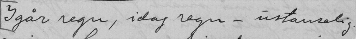Igår regn, idag regn - ustanselig.
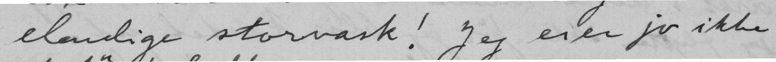elendige storvask! Jeg eier jo ikke
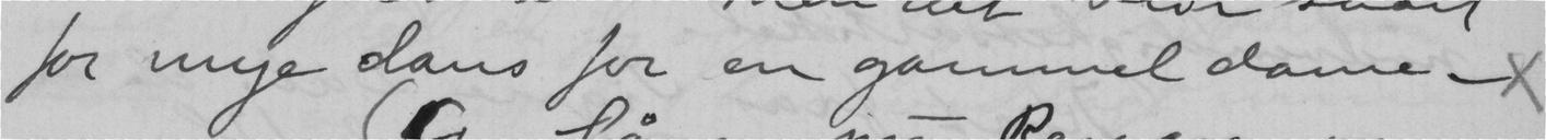for mye dans for en gammel dame - x
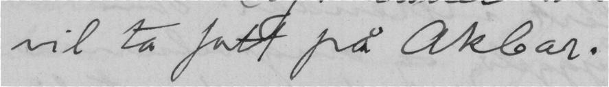vil ta fatt på Akbar.
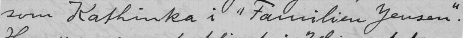som Kathinka i "Familien Jensen".
